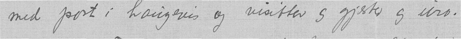med post i haugevis og visiter og gjester og uro.
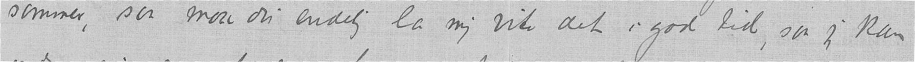sommer, saa maa du endelig la mig vite det i god tid, saa jeg kan
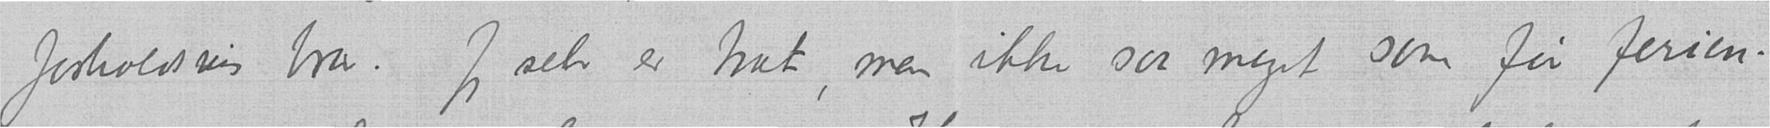forholdsvis bra. Jeg selv er træt, men ikke saa meget som før ferien.
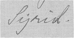Sigrid.
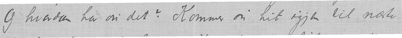Og hvordan har du det? Kommer du hit igjen til næste
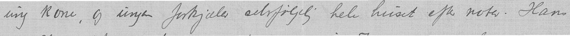ung kone, og ungen forkjæler selvfølgelig hele huset efter noter. Hans
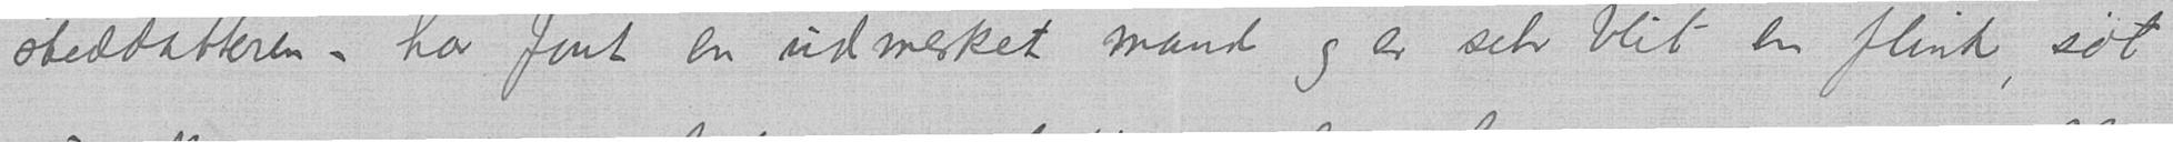steddatteren — har faat en udmerket mand og er selv blit en flink, søt
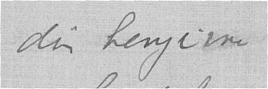din hengivne
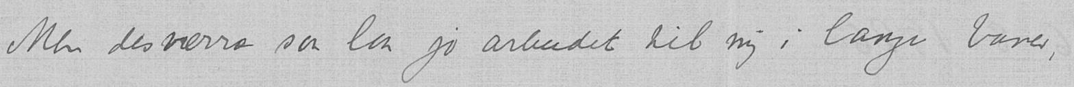Men desværre saa laa jo arbeidet til mig i lange baner,
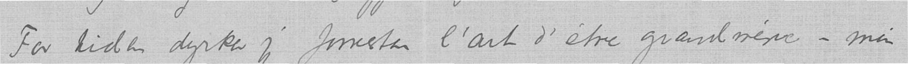For tiden dyrker jeg forresten l'art d'être grandmére — min
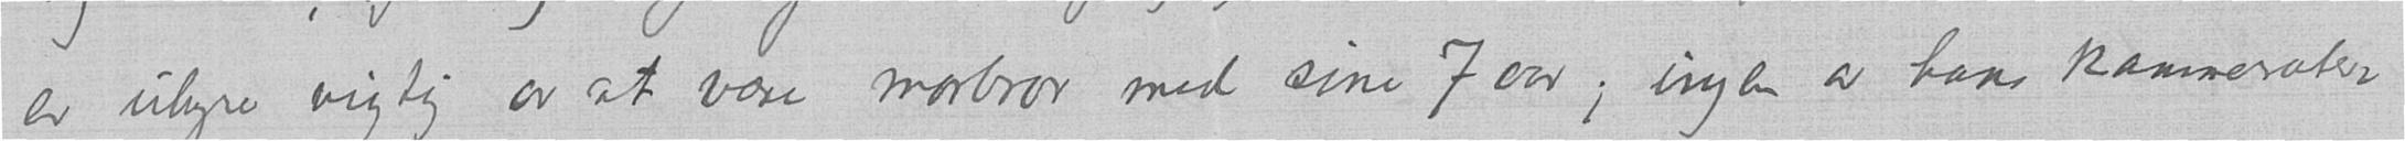er uhyre vigtig av at være morbror med sine 7 aar; ingen av hans kammerater
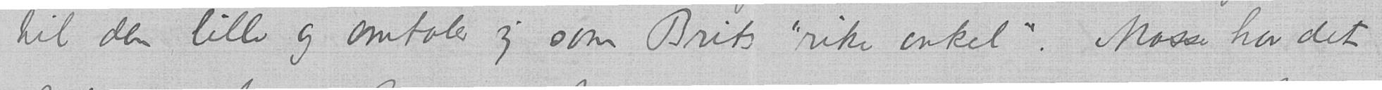til den lille og omtaler sig som Brits "rike onkel". Mosse har det
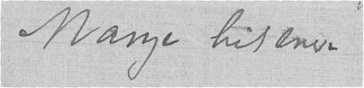Mange hilsener
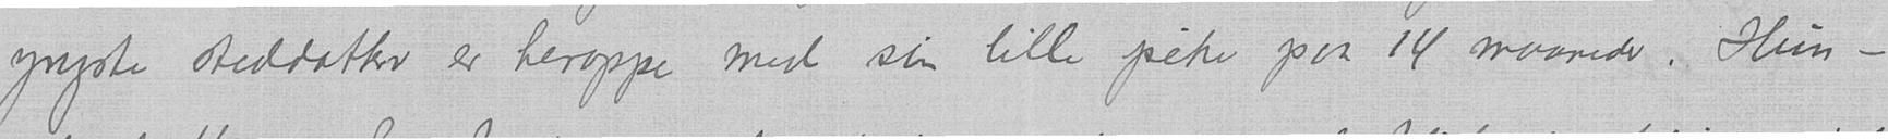yngste steddatter er heroppe med sin lille pike paa 14 maaneder. Hun —
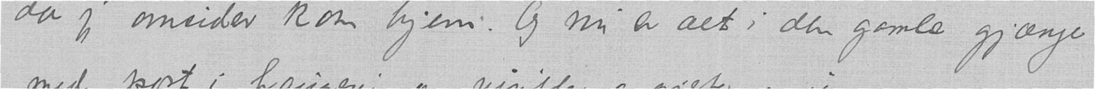da jeg omsider kom hjem. Og nu er alt i den gamle gjænge
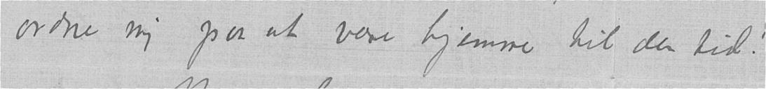ordne mig paa at være hjemme til den tid.
